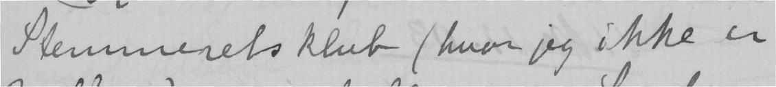Stemmerets klub (hvor jeg ikke er
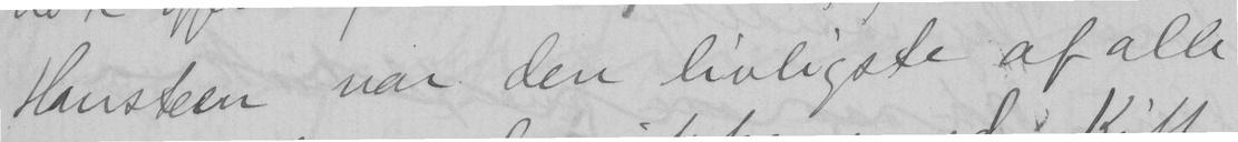Hansteen var den livligste af alle
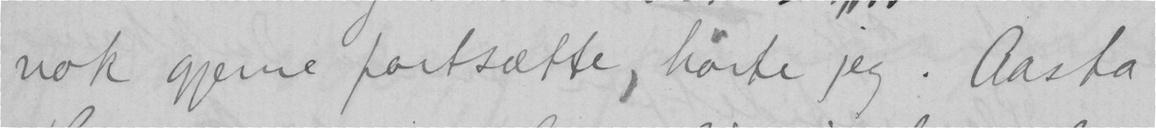nok gjerne fortsætte, hørte jeg. Aasta
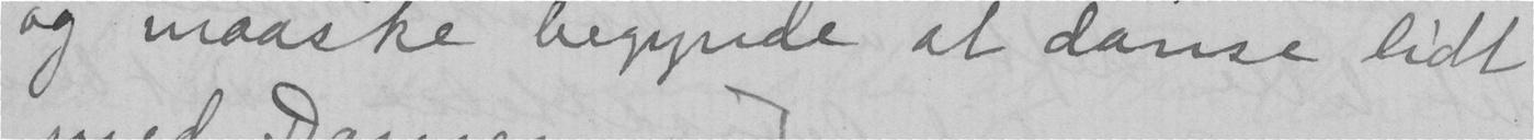og maaske begynde at danse lidt
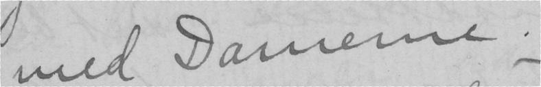med Damerne.
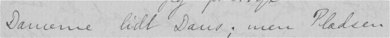Damerne lidt Dans; men Pladsen
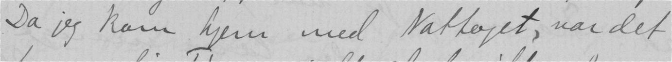Da jeg kom hjem med Nattoget, var det
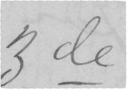3de
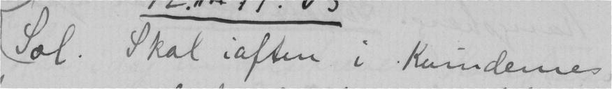Sol. Skal iaften i Kvindernes.
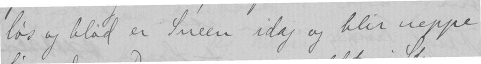løs og blød er Sneen idag og blir neppe
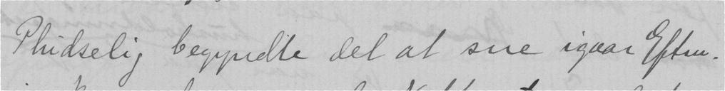Pludselig begyndte det at sne igaar efter.
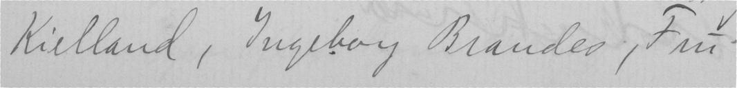Kielland, Ingeborg Brandes, Fru
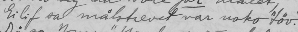Eilif så målstrevet var noko "töv".
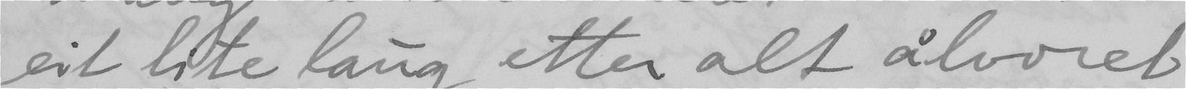eit lite laug etter alt ålvoret
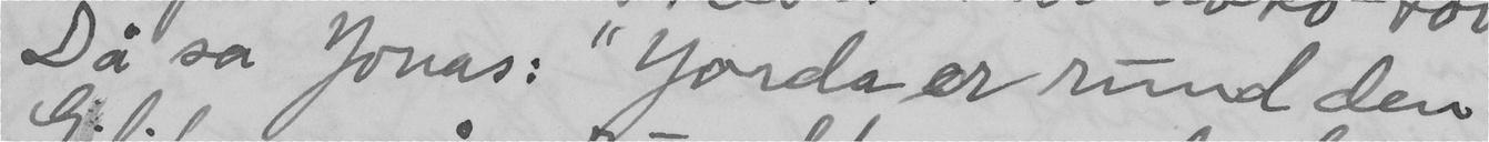Då sa Jonas: "Jorda er rund den
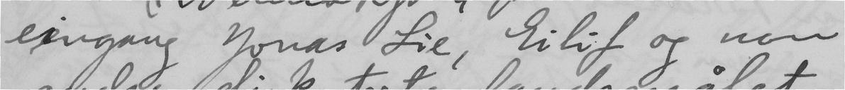eingang Jonas Lie, Eilif og noen
